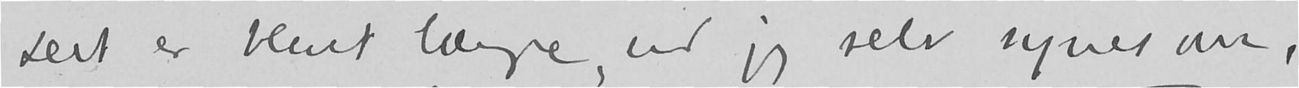Det er blevet længre, end jeg selv synes om,
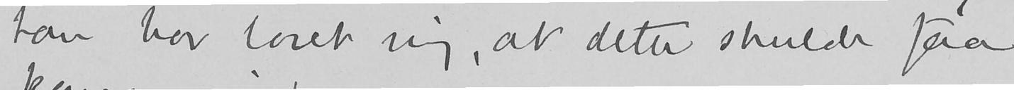han har lovet mig, at dette skulde faa
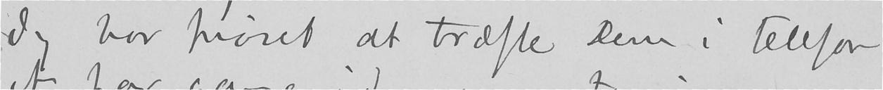Jeg har prøvet at træffe dem i telefon
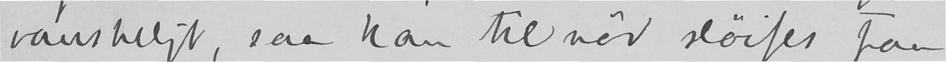vanskeligt, saa kan til nød sløifes paa
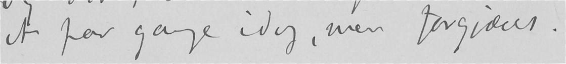et par gange idag, men forgjæves.
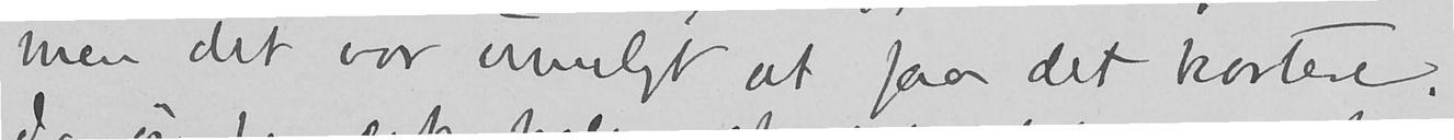men det var umuligt at faa det kortere.
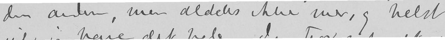den anden, men aldeles ikke mer, og helst
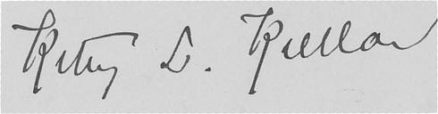Kitty L. Kielland
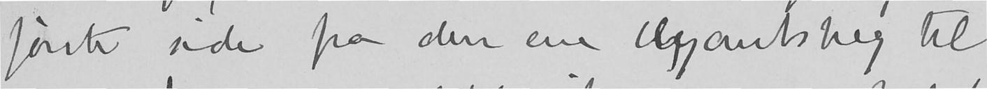første side fra den ene blyantstreg til
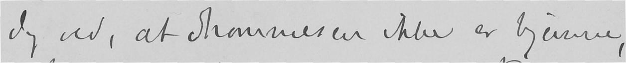Jeg ved, at Thommesen ikke er hjemme,
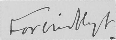Forbindtligt
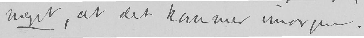meget, at det kommer imorgen.
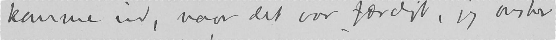komme ud, naar det var færdigt, jeg ønsker
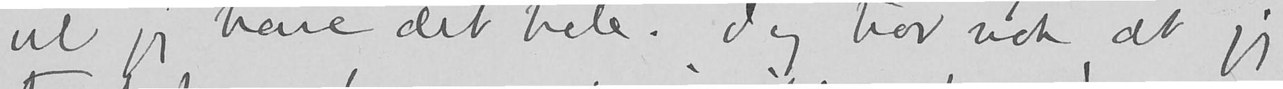vil jeg have det hele. Jeg tror nok at jeg
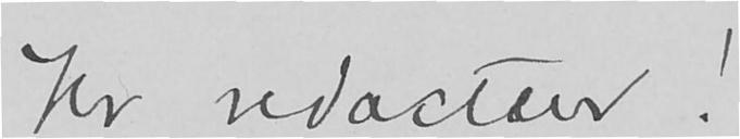Hr redacteur!
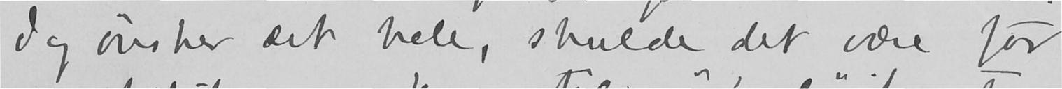Jeg ønsker det hele, skulde det være for
